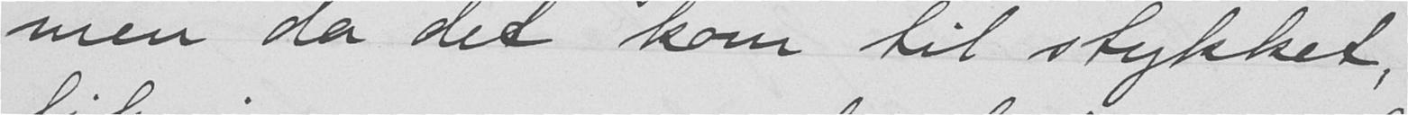men da det kom til stykket,
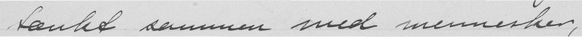tænkt sammen med mennesker,
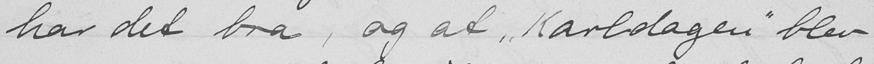har det bra, og at "Karldagen" blev
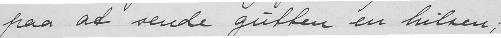paa at sende gutten en hilsen;
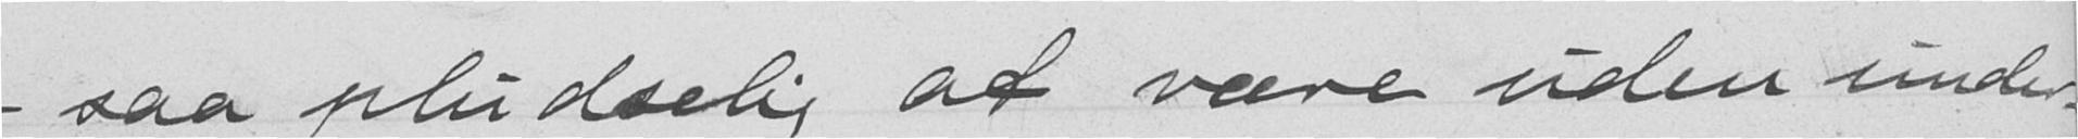- saa pludselig at være uden under-
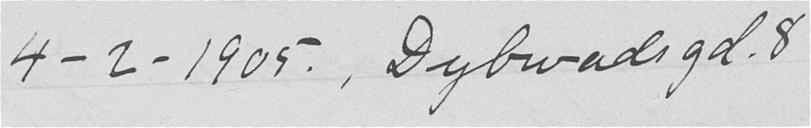4-2-1905, Dybwadsgd. 8
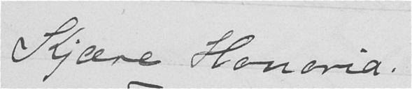Kjære Honoria!
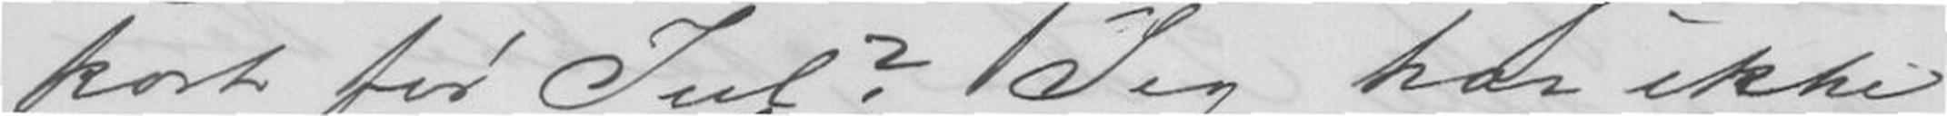kort før Jul? Jeg har ikke
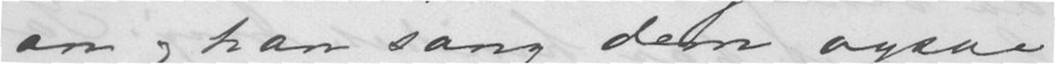an, han sang dem ogsaa
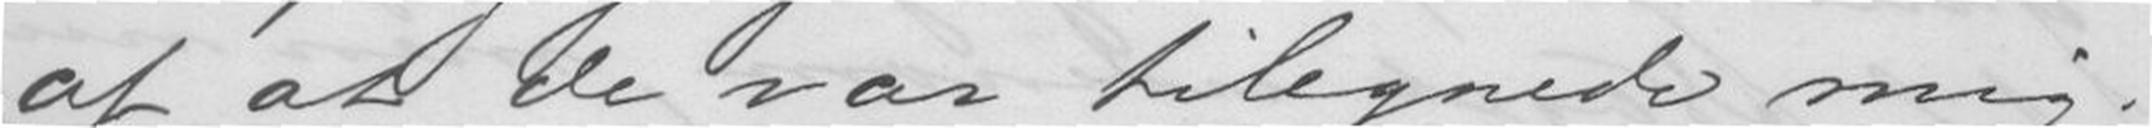af at de var tilegnede mig!
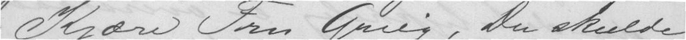- Kjære Fru Grieg, Du skulde
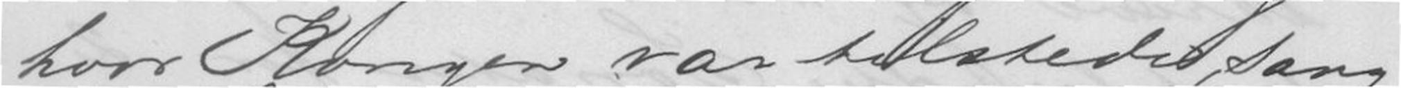hvor Kongen var tilstede, sang
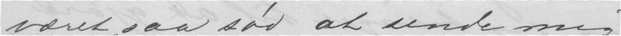været saa sød at sende mig
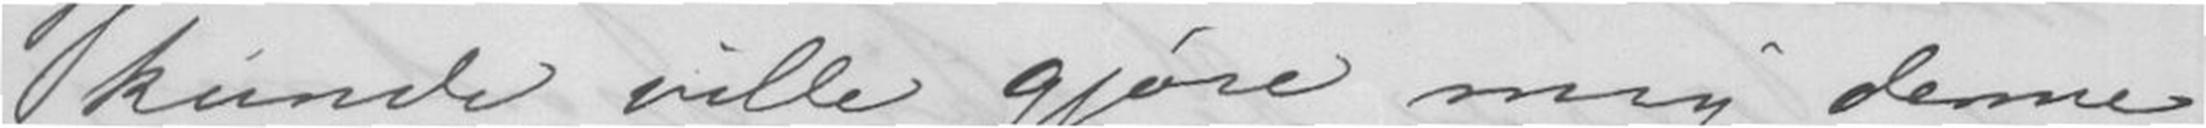kunde ville gjøre mig denne
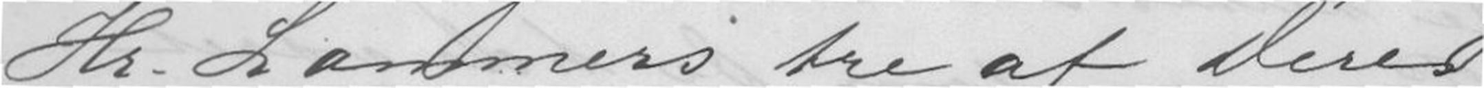Hr Lammers tre af Deres
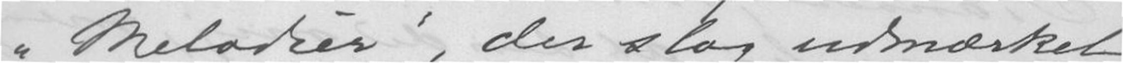Melodier, der slog udmærket
